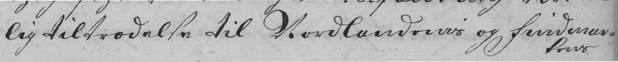lig tiltrædelse til Nordlandenis og Findmar-
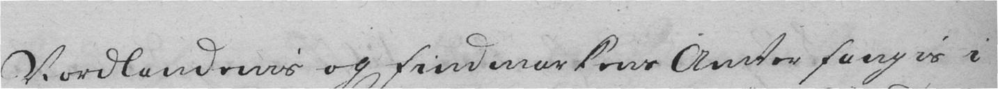Nordlandenis og Findmarkens Amter fangis i
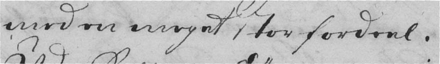med en meget stor fordeel.
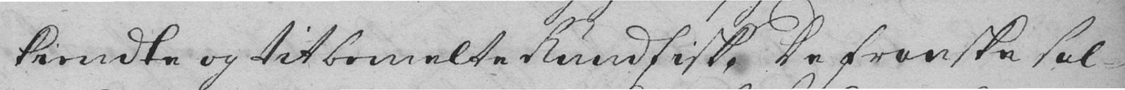kiendte og tit bemelte Rundfisk. De Franske sal-
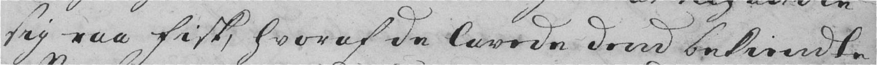sig raa Fisk, hvoraf de lavede dend bekiendte
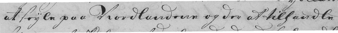at seyle paa Nordlandene og der at tilhandle
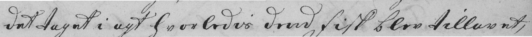det taget i agt hvorledes dend Fisk blev tillavet,
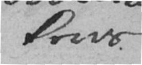kens
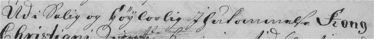Ud i Salig og Høylovlig Ihukommelse Kong
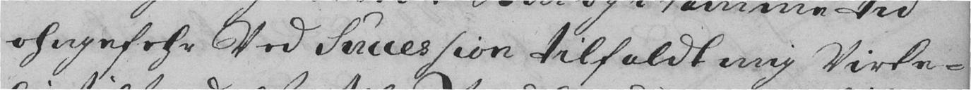ohngefehr Ved Succession tilfaldt mig Virke-
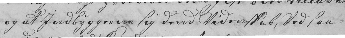og at Indbyggerne sig dend Videnskab, Ved saa
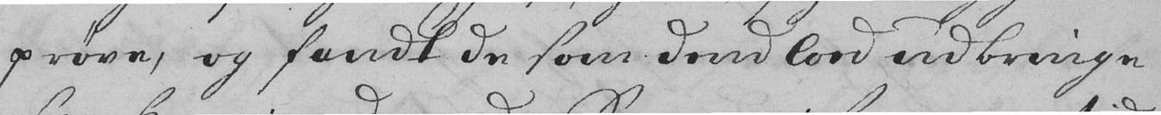prøve, og fandt de som dend loed udbringe
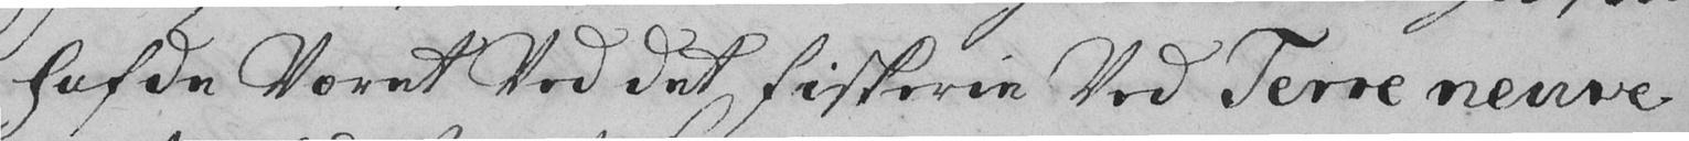hafde Været Ved det Fiskerie ved Terre neuve
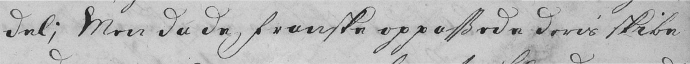del; Men da de franske oppassede deris skibe
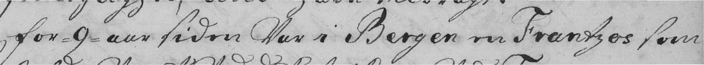For 9 aar siden Var i Bergen en Frantzos som
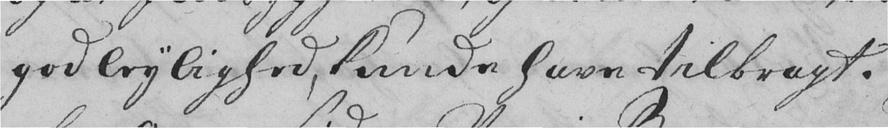god leylighed, kunde have tilbragt.
